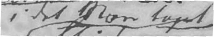i det Store taget
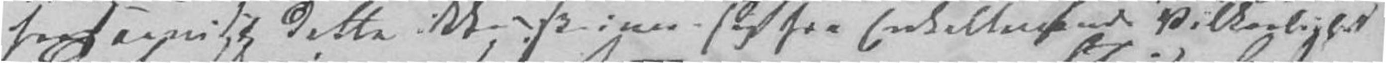forsaavidt dette ikke skriver sig fra Enkeltensende Vilkaarlighed
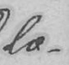læ-
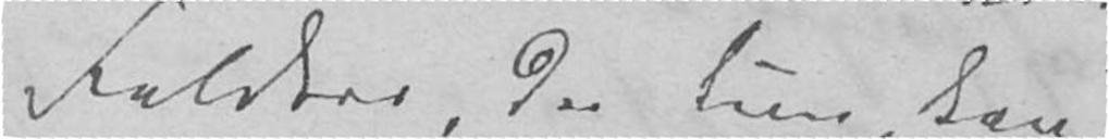Feldter, der kun kan
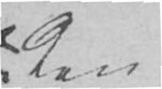Den
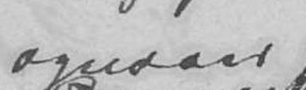opnaaes
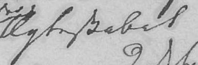Ægteskabet
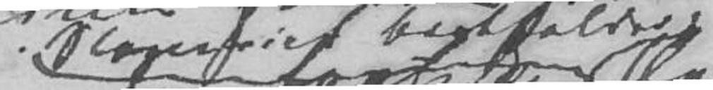Slaveriet bortfalder,
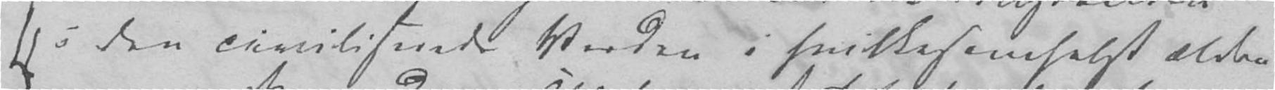i den civiliserede Verden i hvilkesomhelst ældre
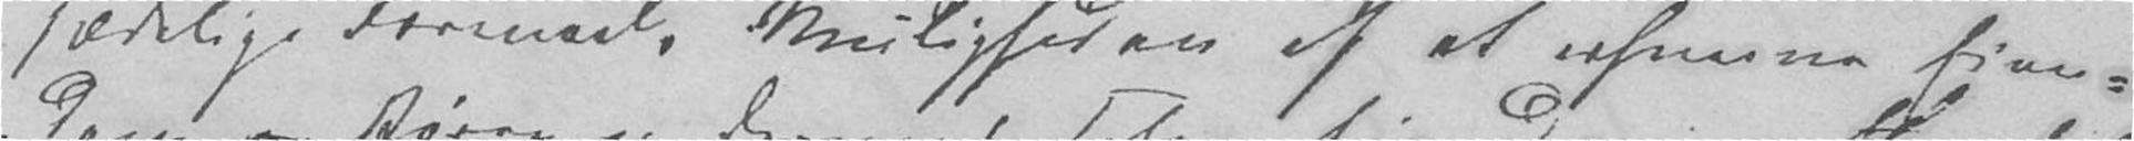sædelige Formaal, Muligheden af at erhverve Eien-
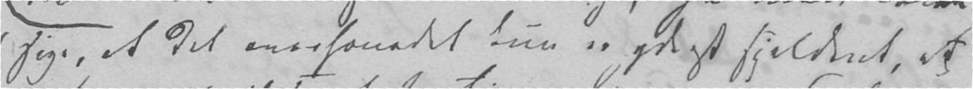sige, at det overhovedet kun er yderst sjeldent, at
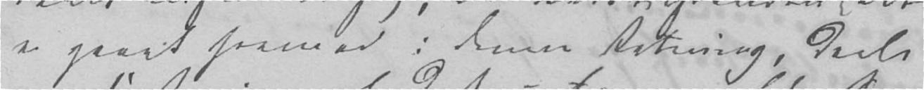er gaaet fremad i denne Retning, deels
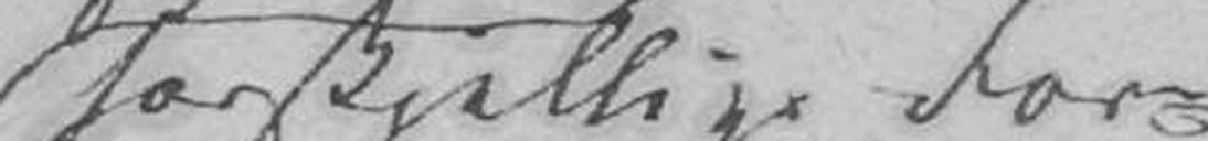forskjellige For-
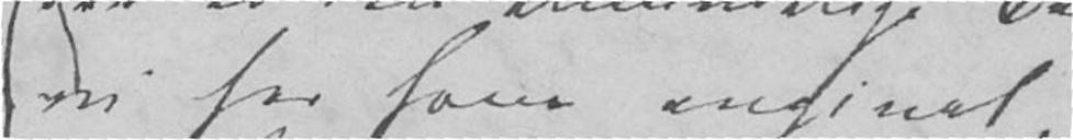vi her have angivet,
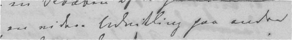en videre Udvikling paa andre
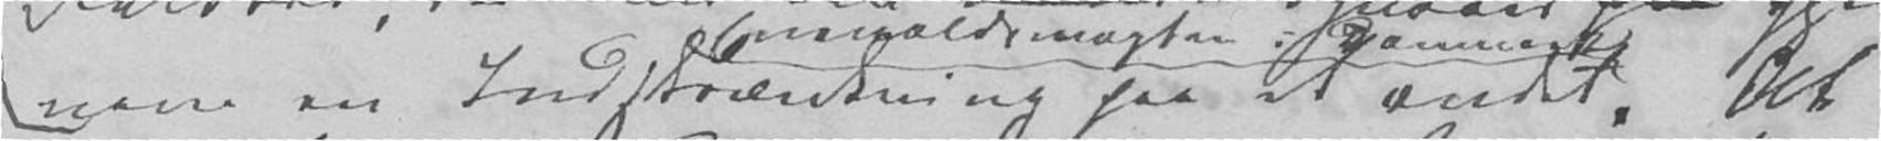nem en Indskrænkning paa et andet. At
